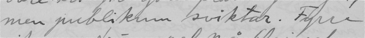men publikum sviktar. Fyrre
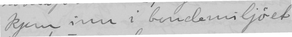kjem inn i bondemiljöet
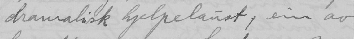dramatisk hjelpelaust, ein av
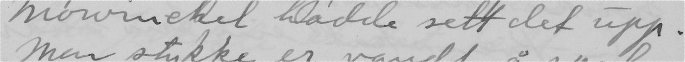Mowinckel hadde sett det upp.
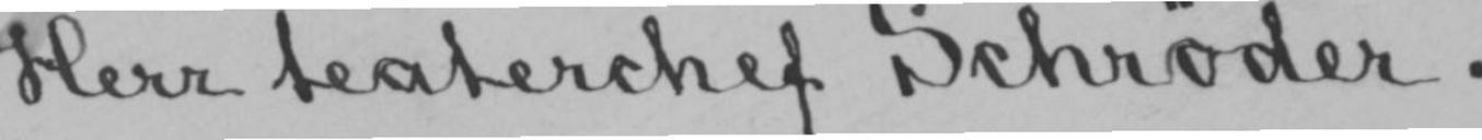Herr teaterchef Schrøder.
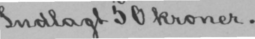Indlagt 50 kroner.
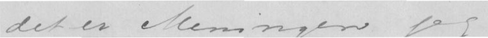det er Meningen jeg
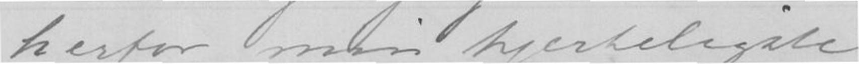herfor min hjerteligste
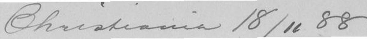Christiania 18/11 88.
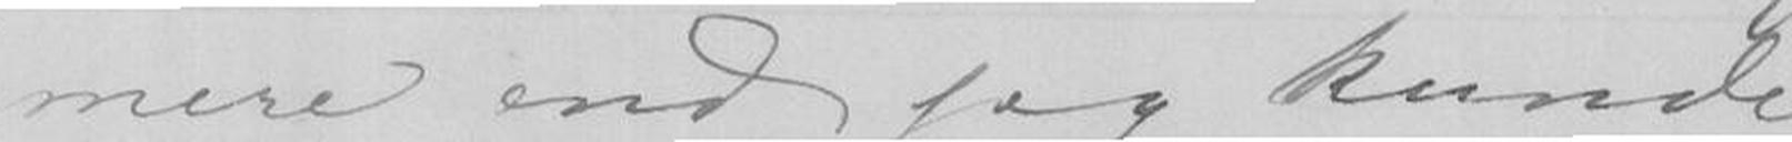mere end jeg kunde
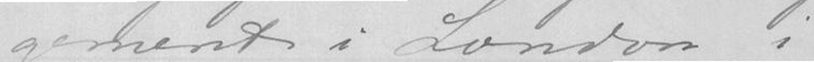gement i London i
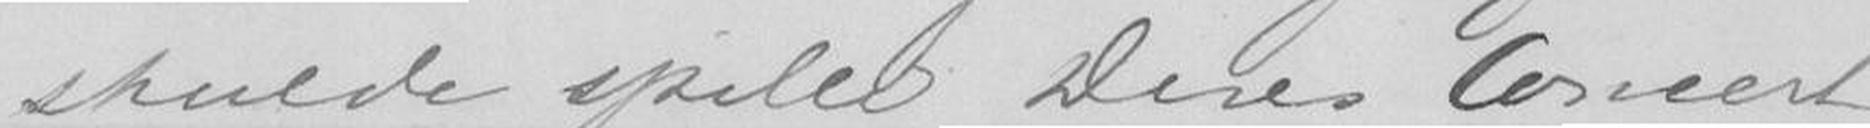skulde spille Deres Concert
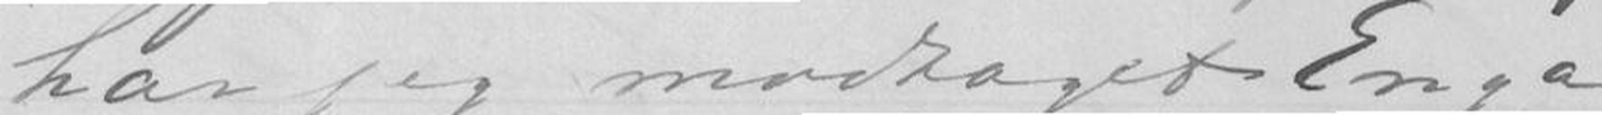har jeg modtaget Enga-
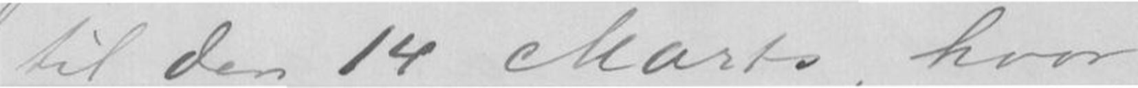til den 14 Marts, hvor
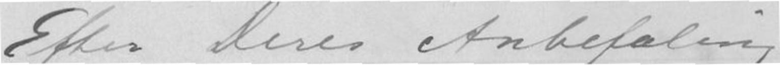Efter Deres Anbefaling
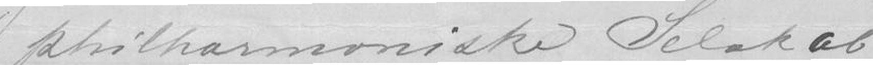philharmoniske Selskab
